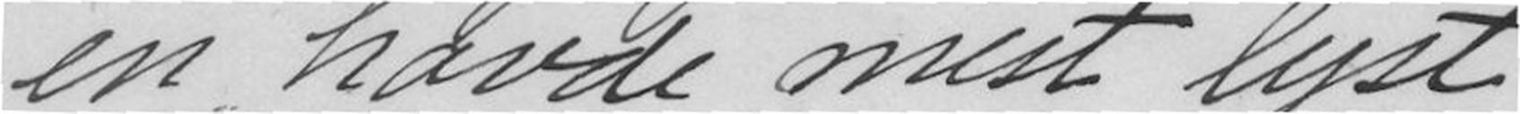en havde mest lyst
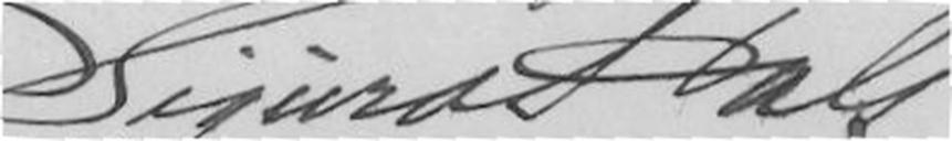Sigurd Hals.
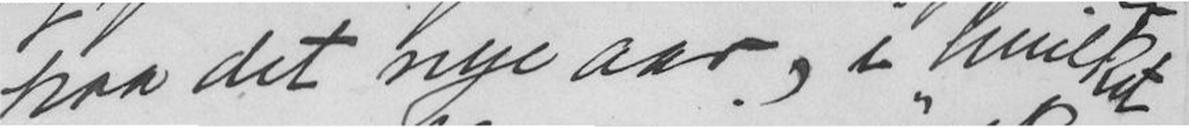paa det nye aar, i hvilket
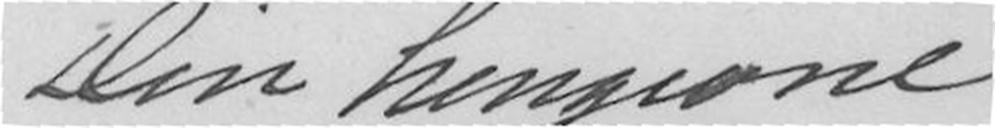Din hengivne
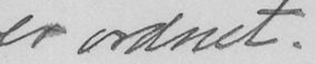er ordnet.
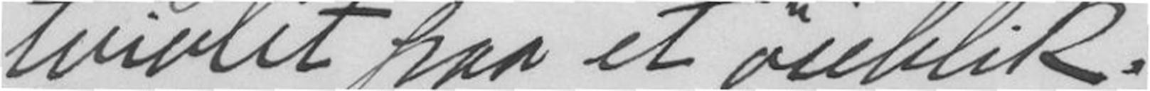tvivlet paa et øieblik.
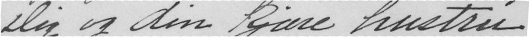Dig og din kjære hustru
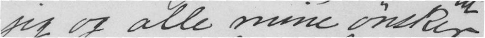jeg og alle mine ønsker
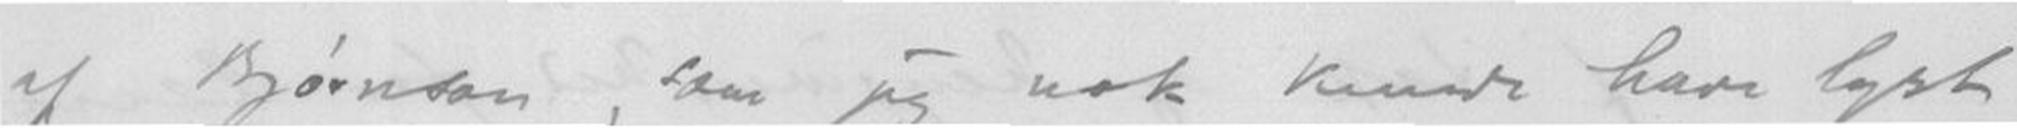af Bjørnson, som jeg nok kunde have lyst
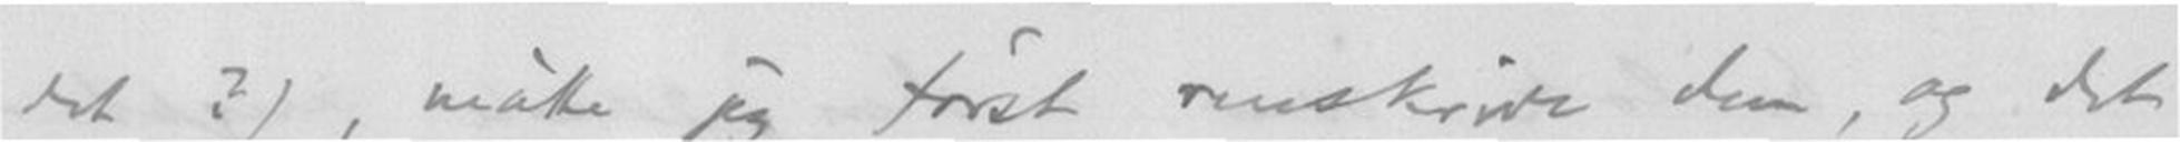det?), måtte jeg først renskrive dem, og det
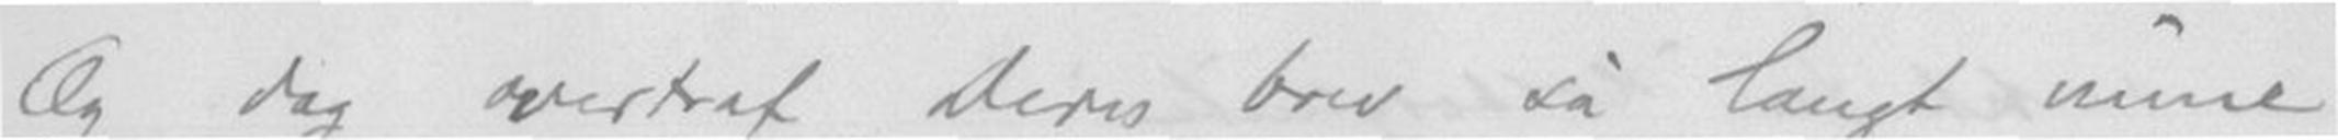Og dog overtraf Deres brev så langt mine
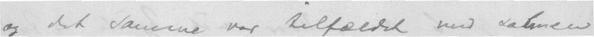og det samme var tilfælde med salmen
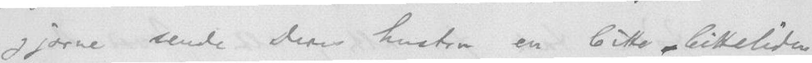gjærne sende Deres hustru en bitte - bitteliden
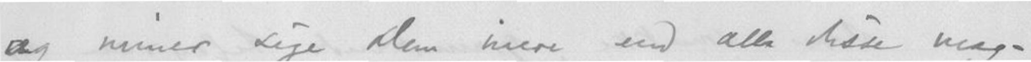og miner sige Dem mere end alle disse mag-
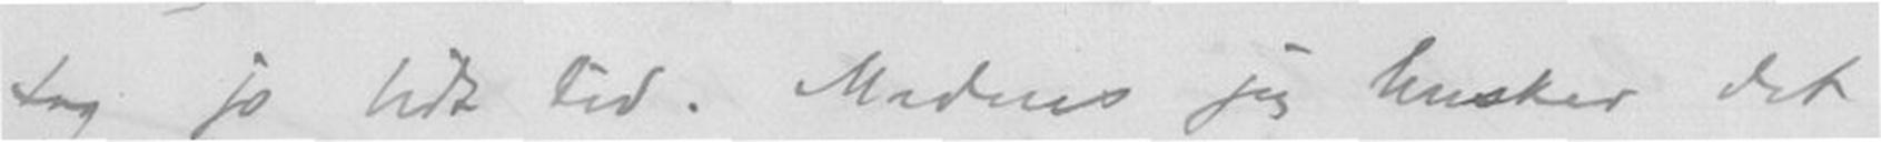tog jo lidt tid. Medens jeg husker det
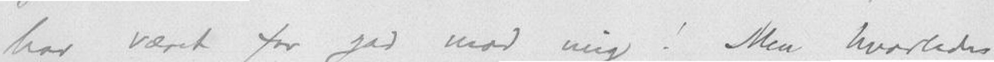har vært for god mod mig! Men hvorledes
